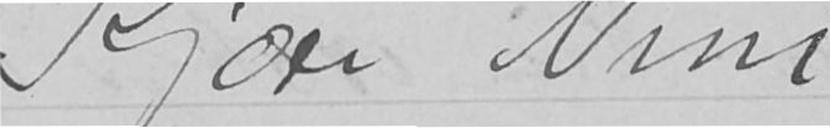Kjære Nini,
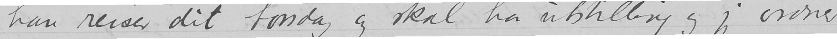han reiser dit torsdag og skal ha utstilling og jeg ordner
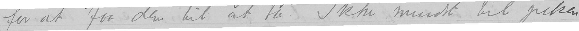for at faa dem til at ta. Ikke mindst til piken.
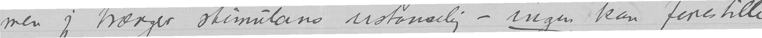men jeg trænger stimulans ustanselig – ingen kan forestille
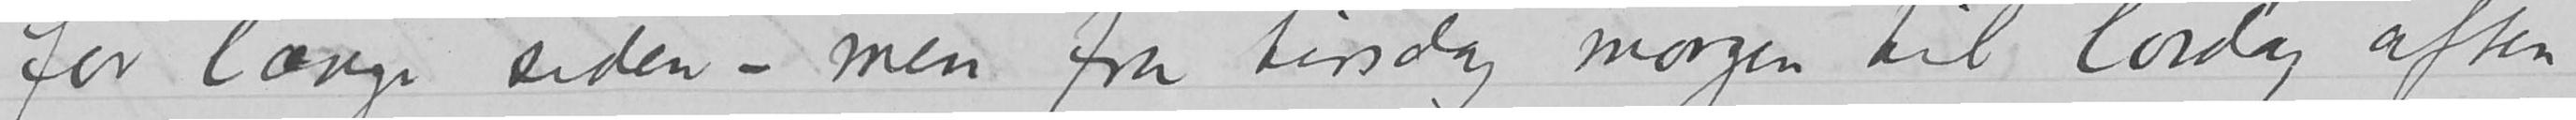for længe siden – men fra tirsdag morgen til lørdag aften
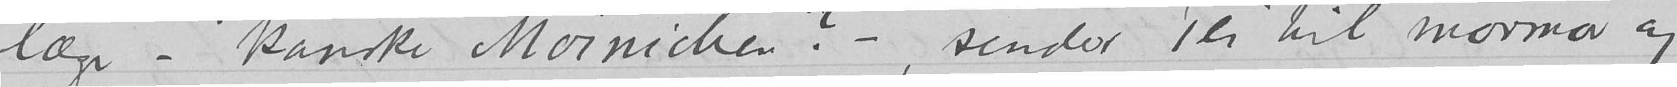læge – kanskje Möinichen? –, sender Pei til mormor og
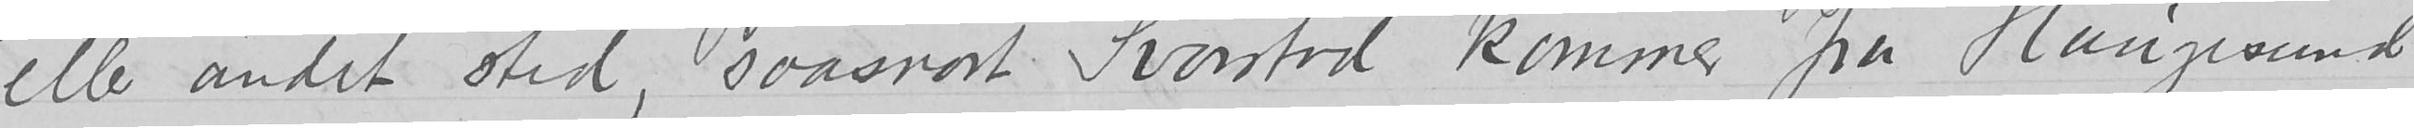eller andet sted, saasnart Svarstad kommer fra Haugesund –
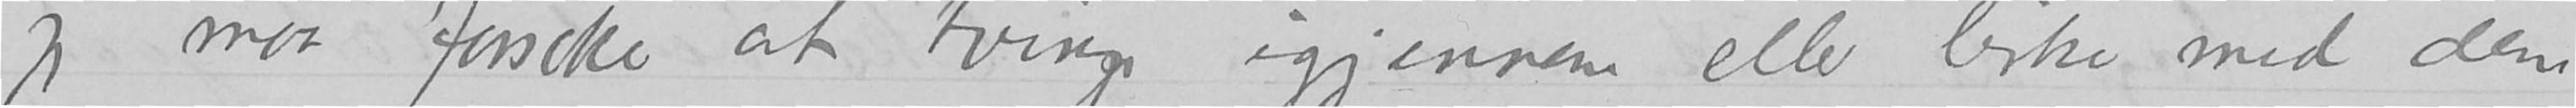jeg maa forsøke at tvinge igjennem eller lirke med dem
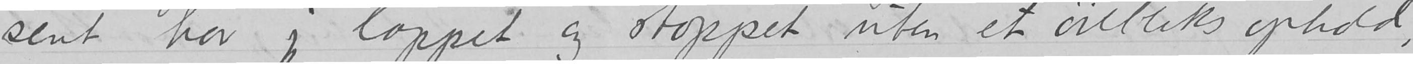sent har jeg lappet og stoppet uten et øiebliks ophold,
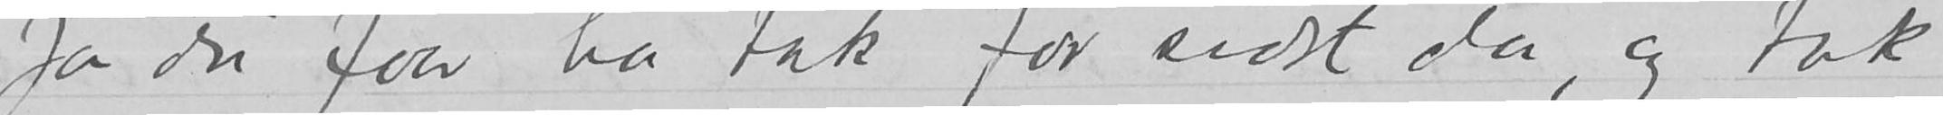Ja du faar ha tak for sidst da, og tak
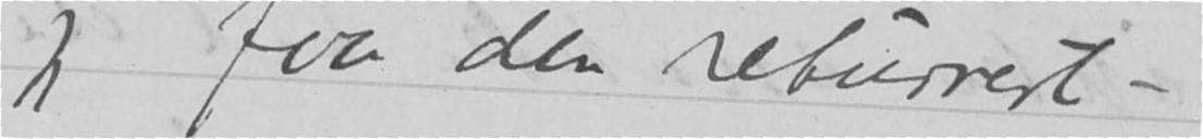jeg faa den returnert-
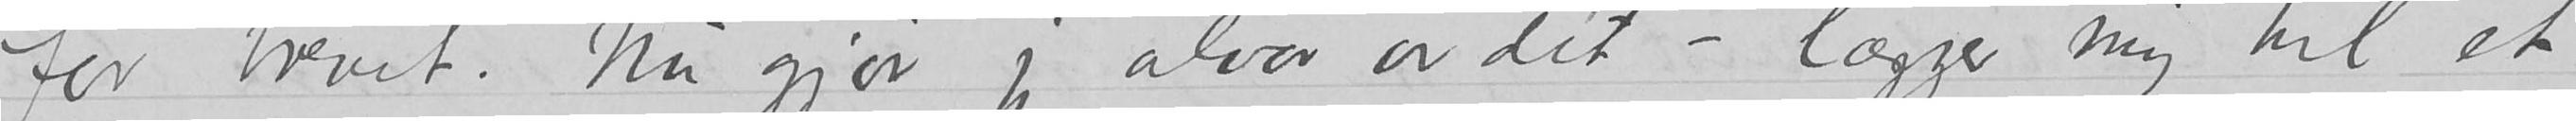for brevet. Nu gjør jeg alvor av det – lægger mig til et
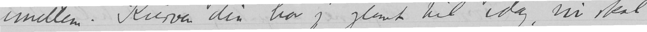imellem. Kurven din har jeg glemt til i dag, nu skal
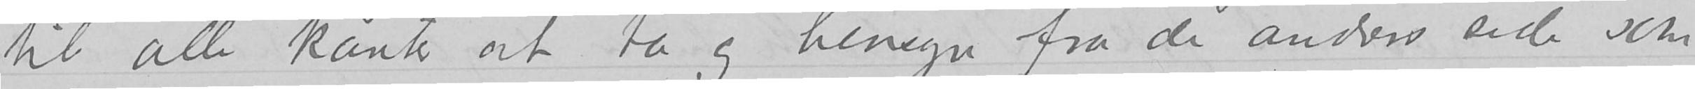til alle kanter at ta og hensyn fra de andres side som
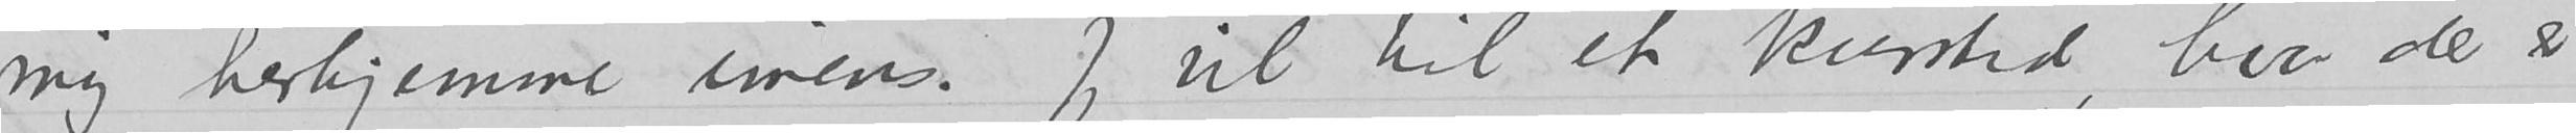mig herhjemme imens. Jeg vil til et kursted, hvor der er
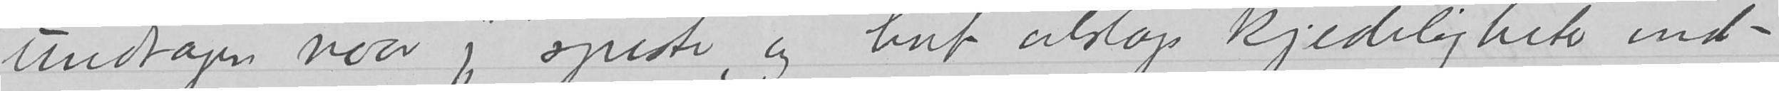undtagen naar jeg spiste, og hat alslags kjedeligheter ind-
